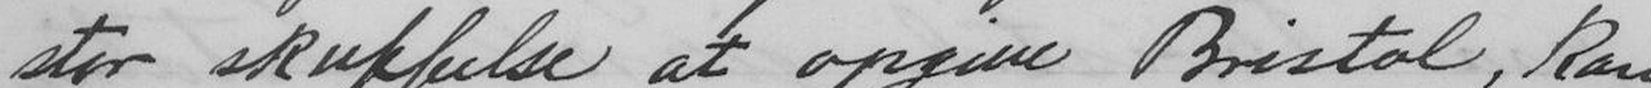stor skuffelse at opgive Bristol, kan
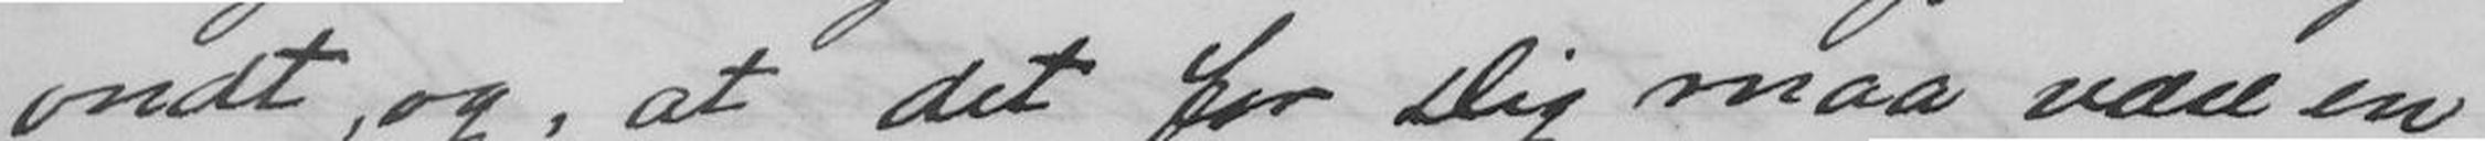ondt, og, at det for Dig maa være en
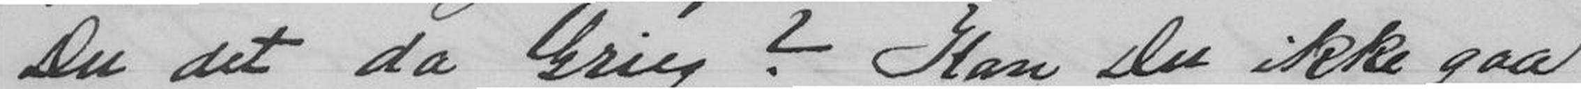Du det da Grieg? Kan Du ikke gaa
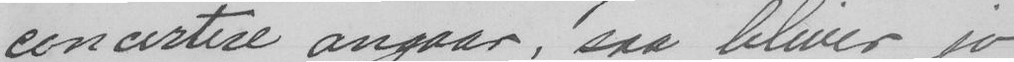concertere angaar, saa bliver jo
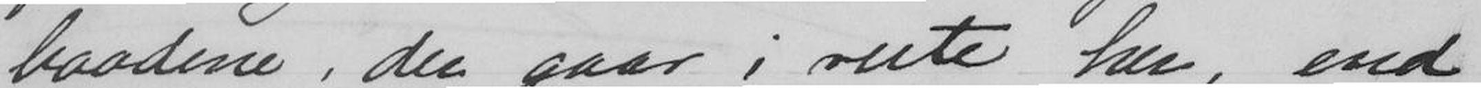baadene, der gaar i rute her, end
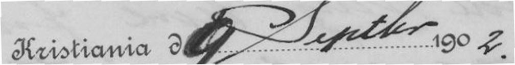Kristiania d. 9 Septbr 1902.
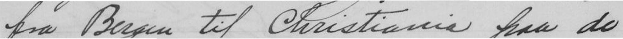fra Bergen til Christiania paa de
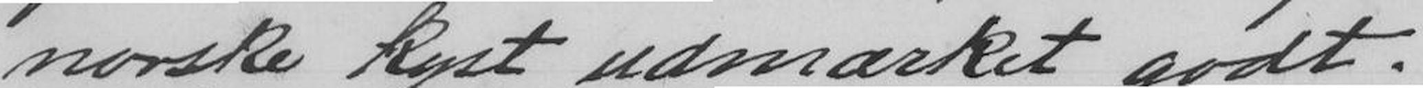norske kyst udmærket godt.
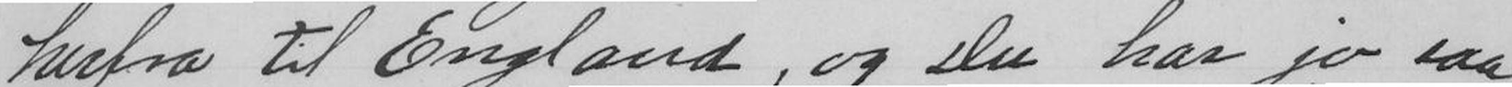herfra til England, og Du har jo saa
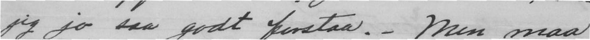jeg jo saa godt forstaa. - Men maa
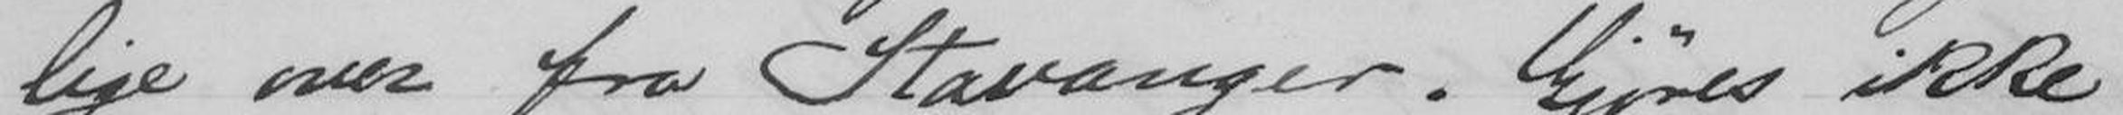lige over fra Stavanger. Gjøres ikke
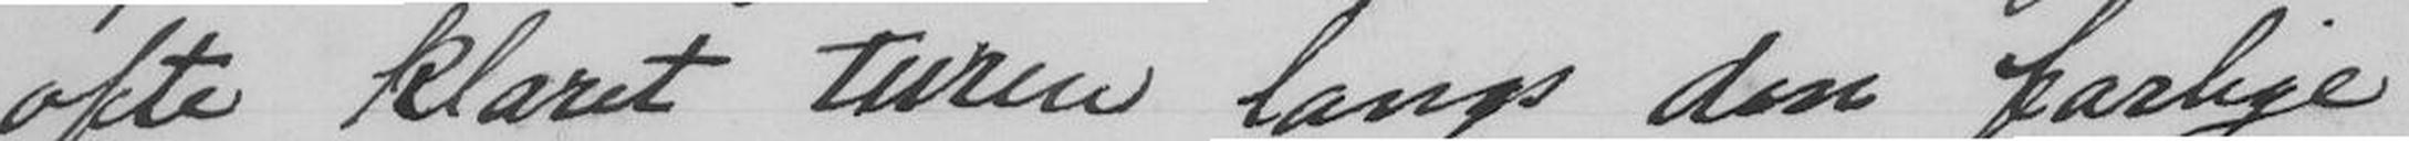ofte klaret turen langs den farlige
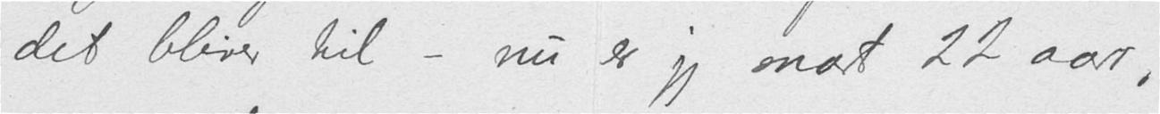det bliver til - nu er jeg snart 22 aar,
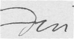Din
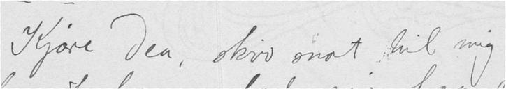Kjære Dea, skriv snart til mig
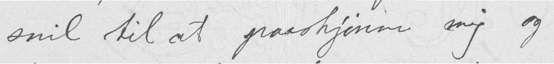snil til at paaskjønne mig og
